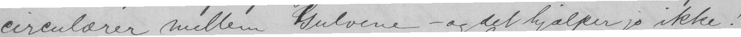circulærer mellem Gulvene - og det hjælper jo ikke!
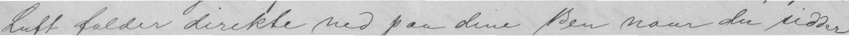Luft falder direkte ned paa dine Ben naar du sidder
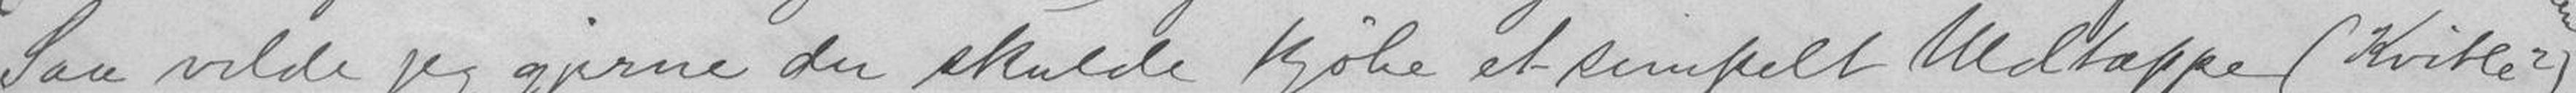Saa vilde jeg gjerne du skulde kjøbe et simpelt Uldtæppe (Kvitle?)
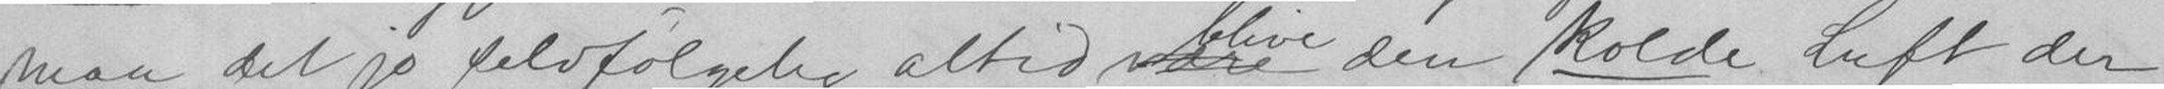maa det jo selvfølgelig altid være blive den kolde Luft der
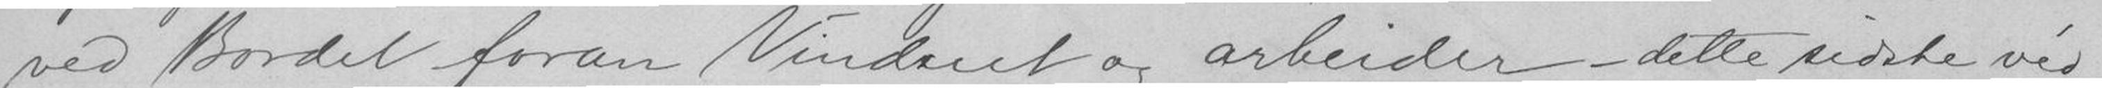ved Bordet foran Vinduet og arbeider - dette sidste véd
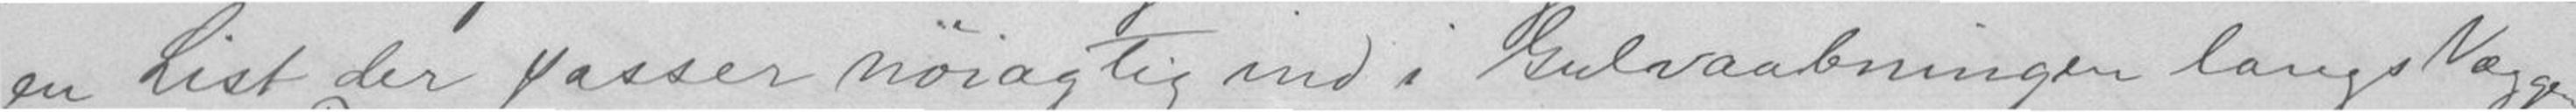en List der passer nøiagitg ind i Gulvaabningen langs Væggen
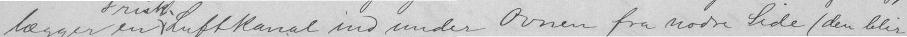lægger en Luftkanal ind under Ovnen fra nodre Side (den blir
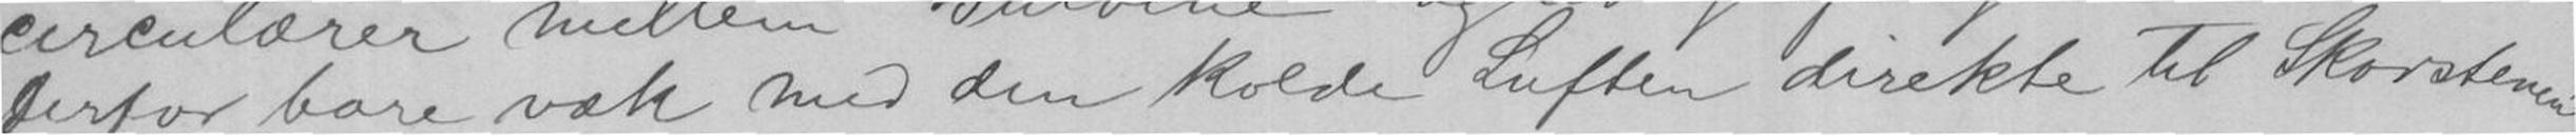Derfor bare væk med den kolde Luften direkte til Skorstenen
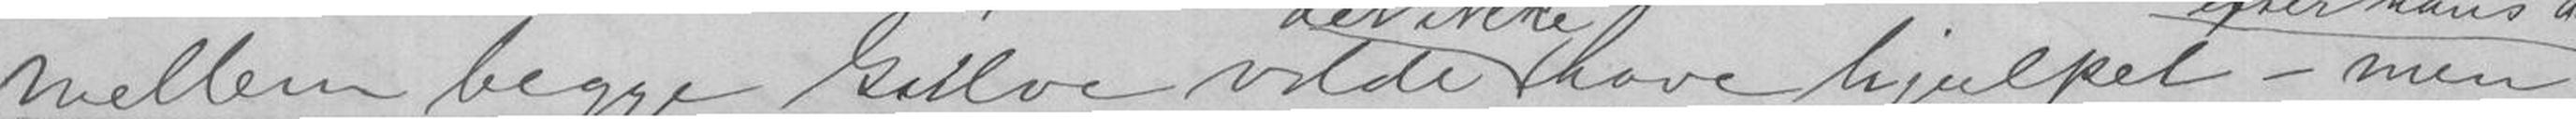mellem begge Gulve vilde have hjulpet - men
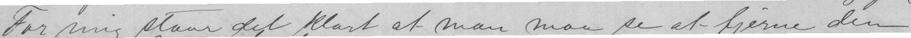For mig staar det klart at man maa se at fjerne den
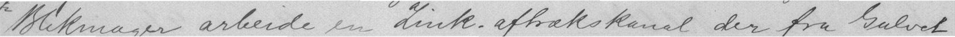Blekmager arbeide en Zink-aftrækskanal der fra Gulvet
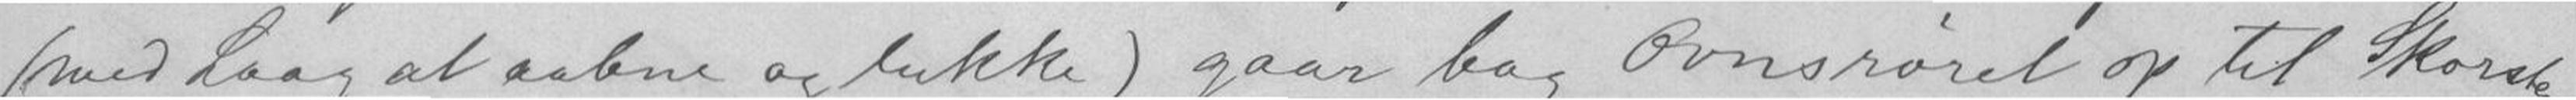(med Laag at aabne og lukke) gaar bag Ovnsrøret op til Skorstenen
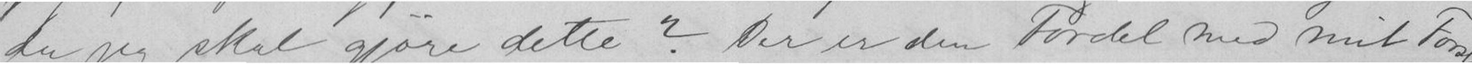du jeg skal gjøre dette? Der er den Fordel med mit Forslag
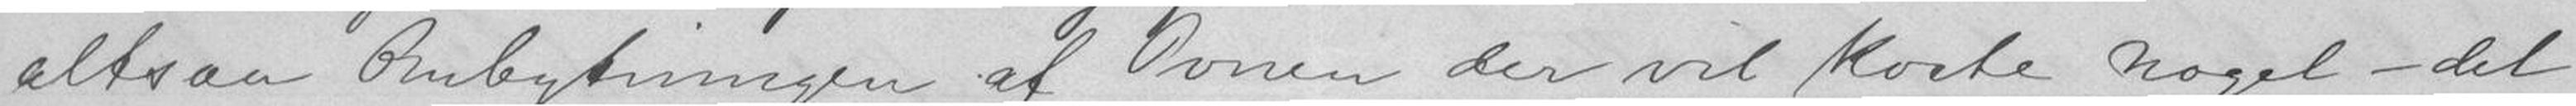altsaa Ombytningen af Ovnen der vil koste noget - det
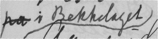i Bekkelaget
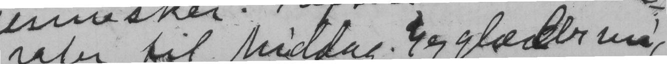rater til Miadag. Jeg glæder mig
